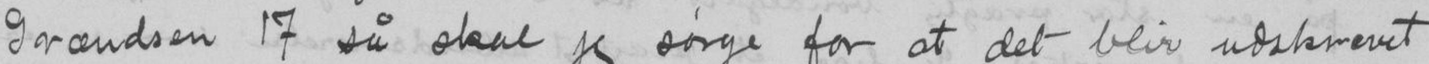Grændsen 17 så skal jeg sørge for at det blir udskrevet.
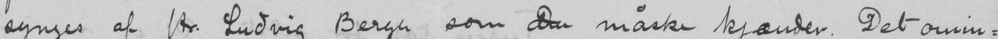synes af Hr. Ludvig Bergh som Du måske kjænder. Det omin-
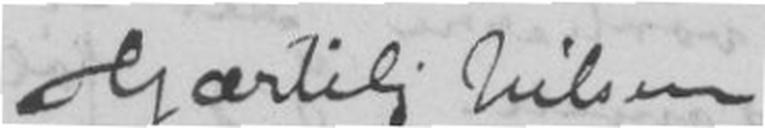Hjærtelig hilsen
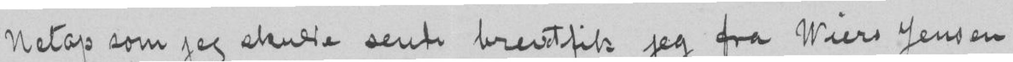Netop som jeg skulde sende brevet fik jeg fra Wiers Jensen
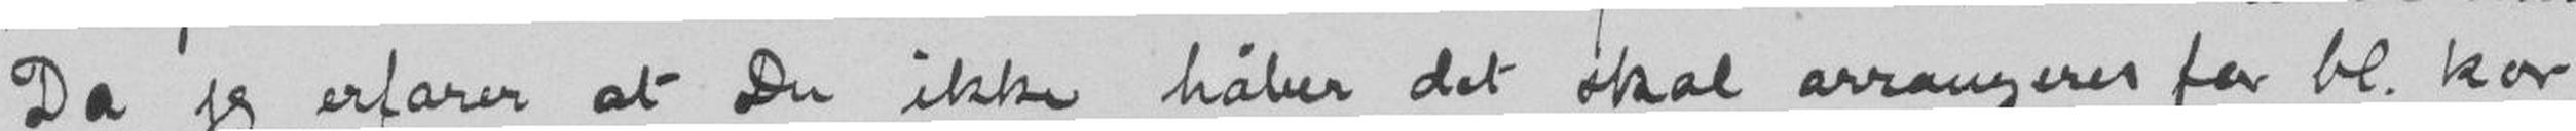Da jeg erfarer at Du ikke håber det skal arrangeres for bl. kor
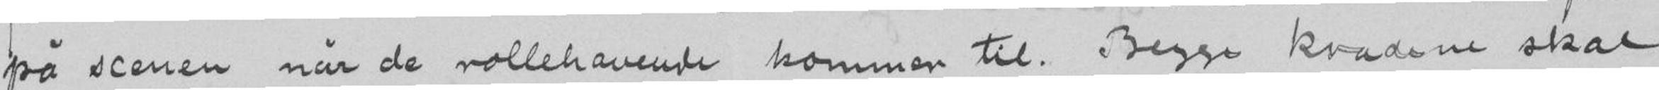på scenen når de rollehavende kommer til. Begge kvadene skal
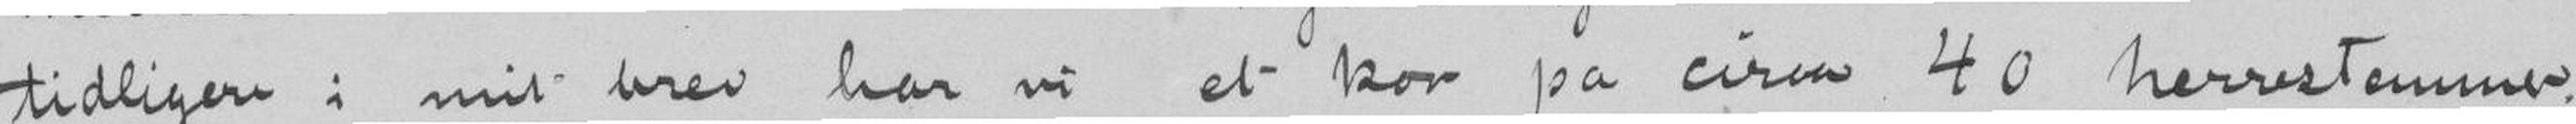tidligere i mit brev har vi et kor på circa 40 herrestemmer.
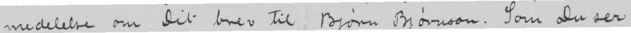medelelse om Dit brev til Bjørn Bjørnson. Som Du ser
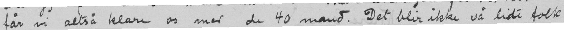får vi altså klare os med de 40 mand. Det blir ikke så lide folk
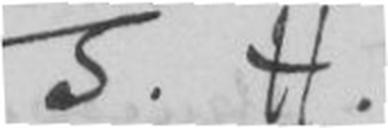J. H.
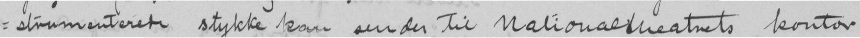strumenterede stykke som sendes til Nationaltheatrets kontor
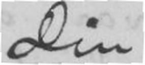Din
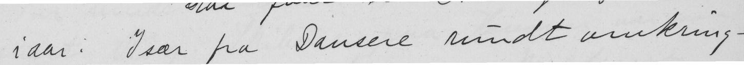iaar. Især fra Dansere rundt omkring-
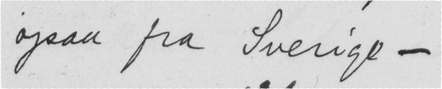ogsaa fra Sverige -
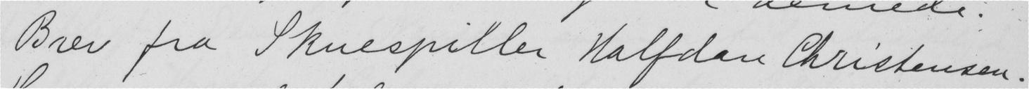Brev fra Skuespiller Halfdan Christensen.
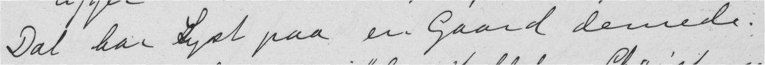Dal har Lyst paa en Gaard dernede.
